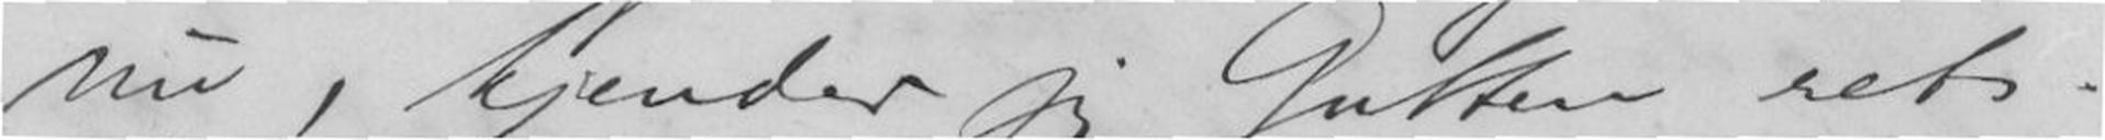nu, kjender jeg Gutten ret.
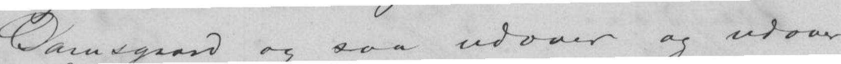Damsgaard og saa udover og udover
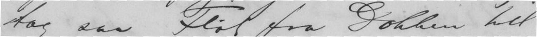tog saa Fløt fra Dokken til
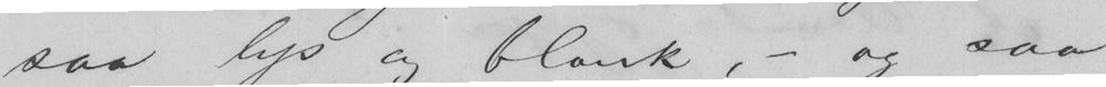saa lys og blank, - og saa
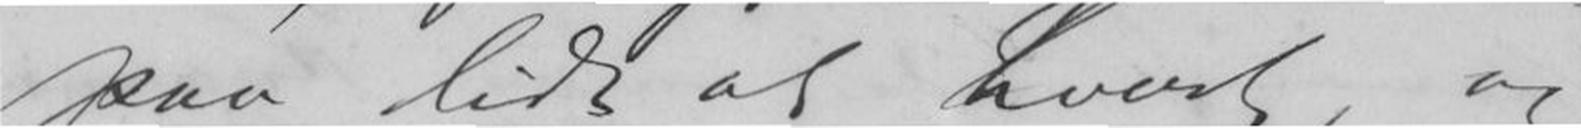paa lidt af hvert, og
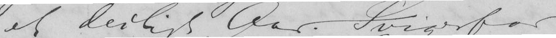et deiligt Aar. Svigerfar
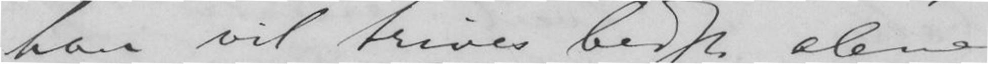han vil trives bedst alene.
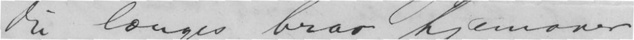du længes brav hjemover
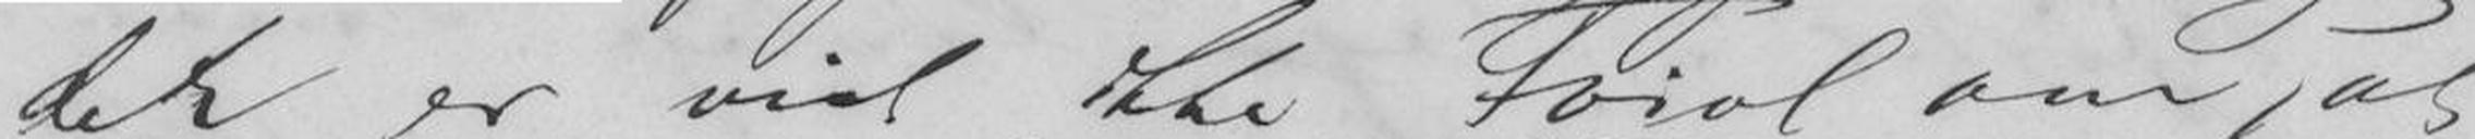der er vist ikke Tvivl om, at
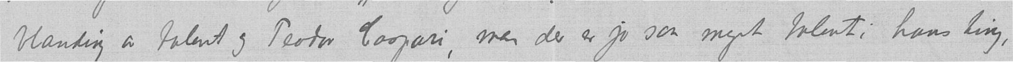blanding av talent og Teodor Caspari, men der er jo saa meget talent i hans ting,
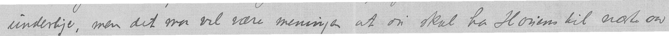underlige, men det maa vel være meningen at du skal ha Houens til næste aar
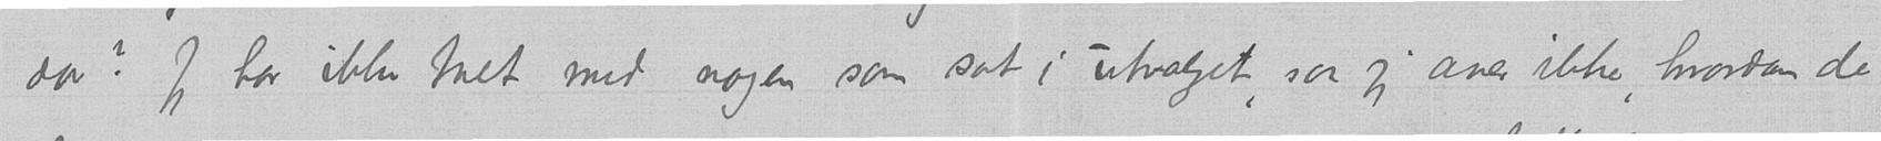da? Jeg har ikke talt med nogen som sat i utvalget, saa jeg aner ikke, hvordan de
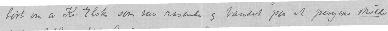hørt om av Kr. Elster som var rasende og bandet paa at pengene skulde
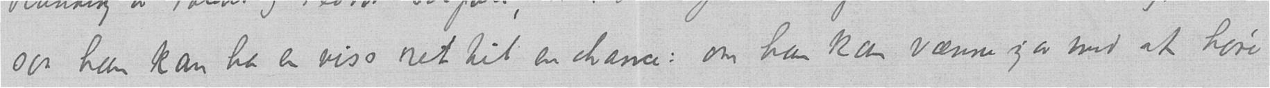saa han kan ha en viss ret til en chance: om han kan vænne sig av med at høre
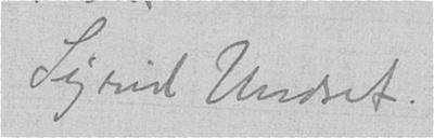Sigrid Undset.
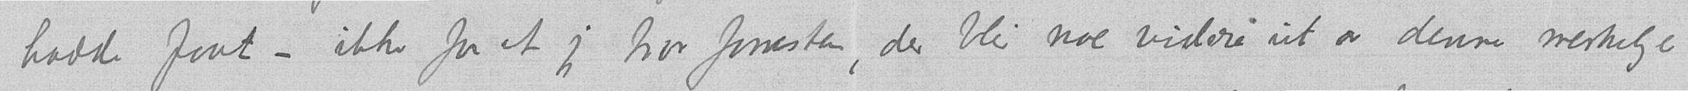hadde faat – ikke for at jeg tror forresten, der blir noe videre ut av denne merkelige
